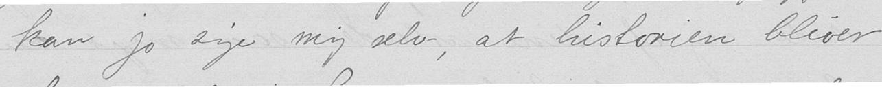kan jo sige mig selv, at historien bliver
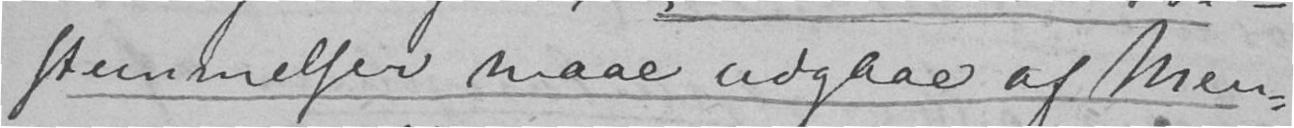stemmelser maae udgaae af Men-
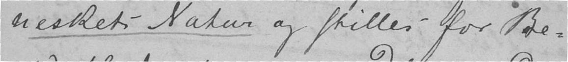neskets Natur og stilles for Be-
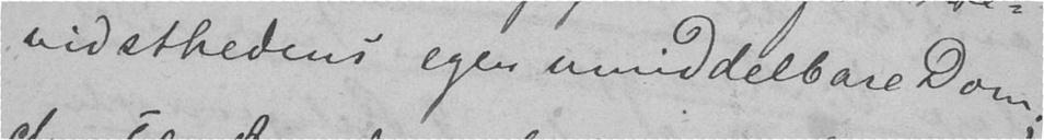vidsthedens egen umiddelbare Dom;
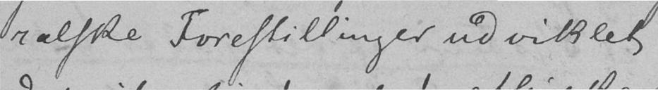ralske Forestillinger udviklet
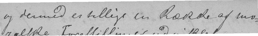og dermed er tillige en Række af mo-
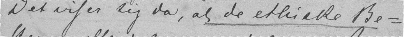Det viser sig da, at de ethiske Be-
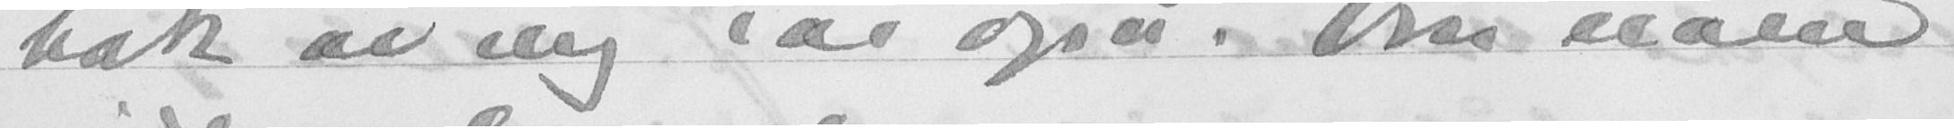bok av mig iår også. Om noen
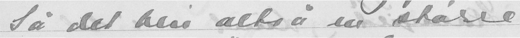Så det blir altså en større
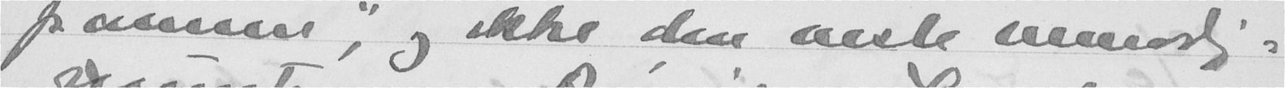famisen; og ikke den neste vemodig-
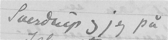Sverdrup og jeg på
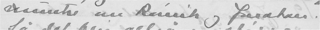vaunke om kunsk, og fonstan.
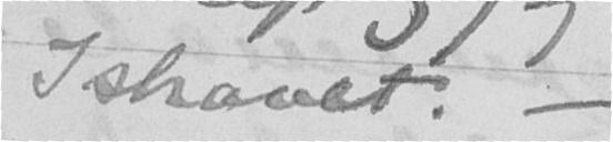Ishavet. -
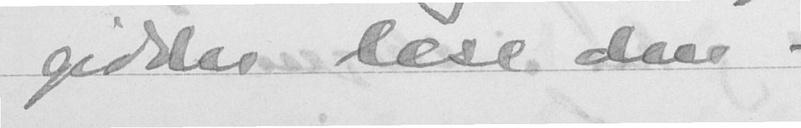gidder lese den.
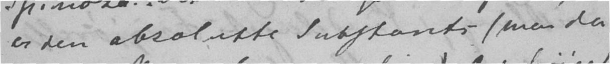er den absolutte Substants (men da
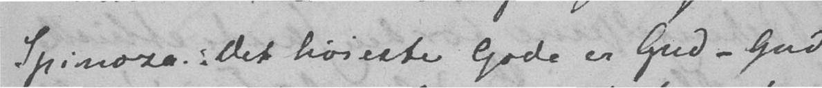Spinoza det høieste Gode er Gud – Gud
